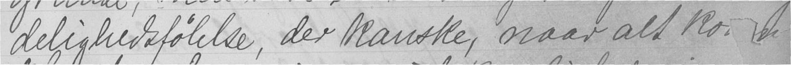delighedsfølelse, der kanske, naar alt kommer
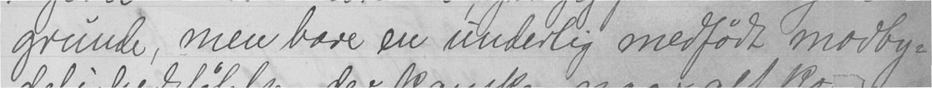grunde, men bare en underlig medfødt modby-
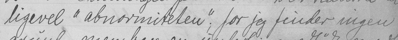ligevel "abnormiteten"; for jeg finder ingen
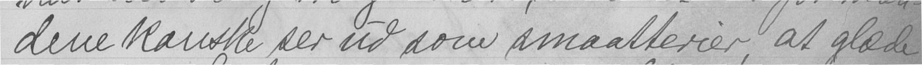dene kanske ser ud som smaatterier, at glæde
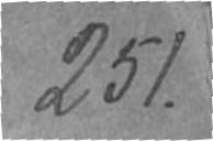251.
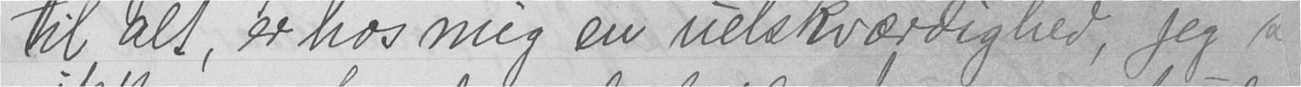til alt, er hos mig en uelskværdighed, jeg a
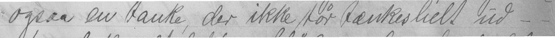ogsaa en tanke, der ikke tør tænkes helt ud - -
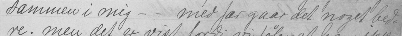sammen i mig - - med far gaar det noget bed-
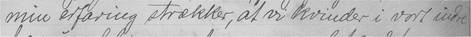min erfaring strækker, at vi kvinder i vort indre
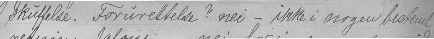skuffelse. Forurettelse? nei - ikke i nogen bestemt
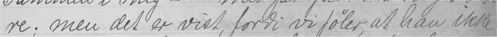re; men det er vist, fordi vi føler, at han ikke
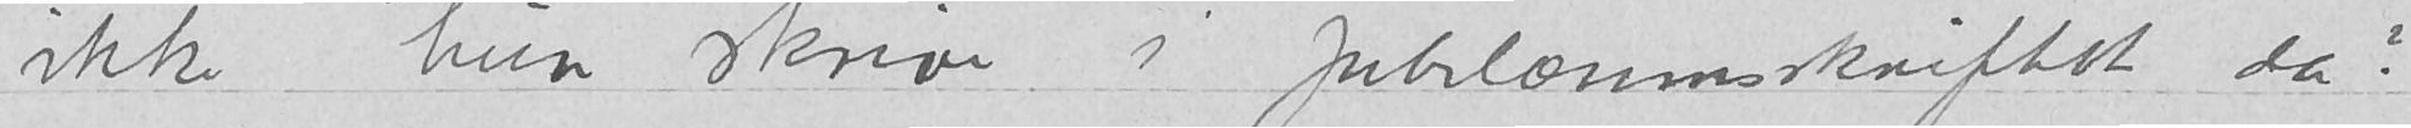ikke hun skrive i jubilæumsskriftet da?
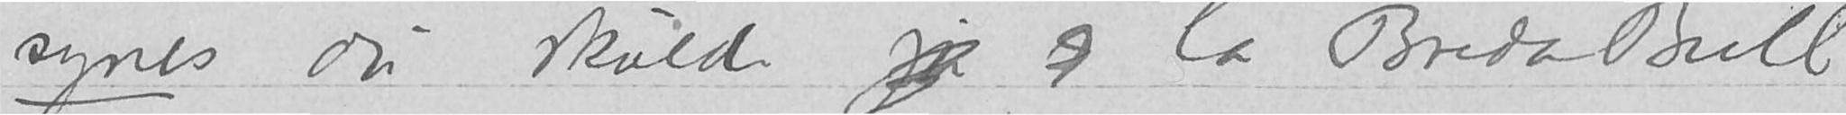Jeg synes du skulde ju ? la Breda Bull
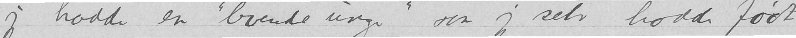jeg hadde en «levende unge» som jeg selv hadde født,
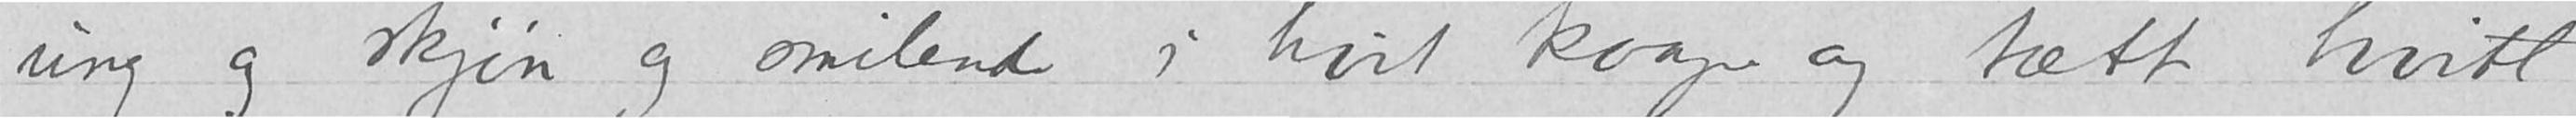ung og skjøn og smilende i hvit kaape og tætt hvitt
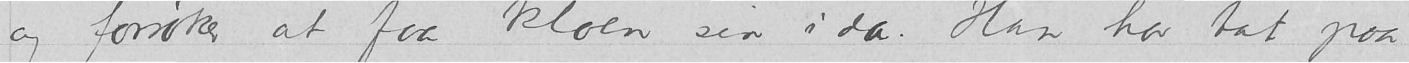og forsøker at faa kloen sin i da. Han har tat paa
